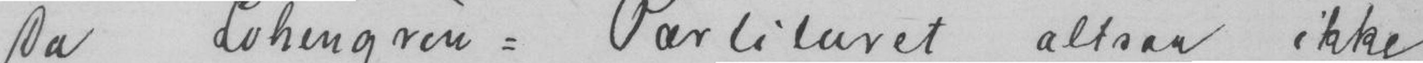Da Lohengrein= Parlituret alsaa ikke
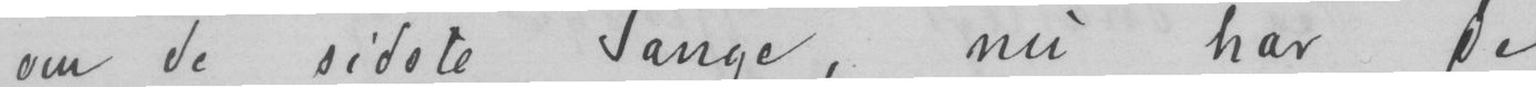om de sidste Sanger, nu har de
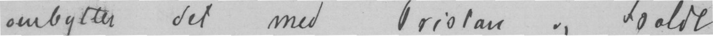ombytter det med Friolan og Igholdt.
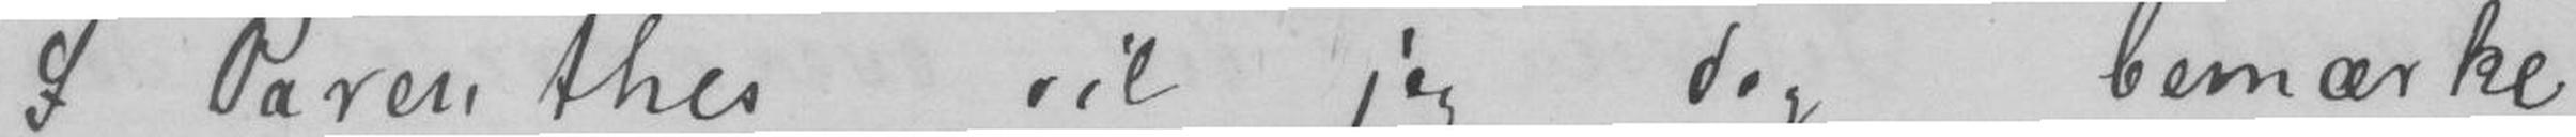I Parenthes vil jeg dog bemærke,
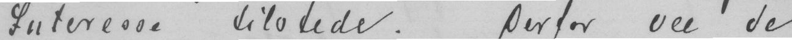Interesse tilstede. Derfor vee de
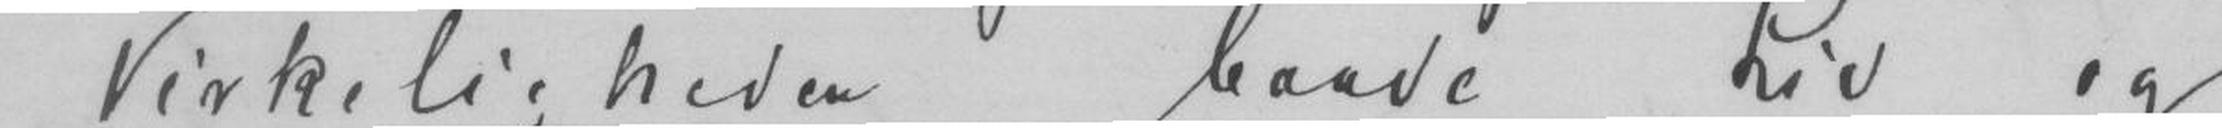i virkeligheden baade Liv og
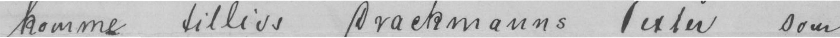komme tillids Drackmanns Fetter, som
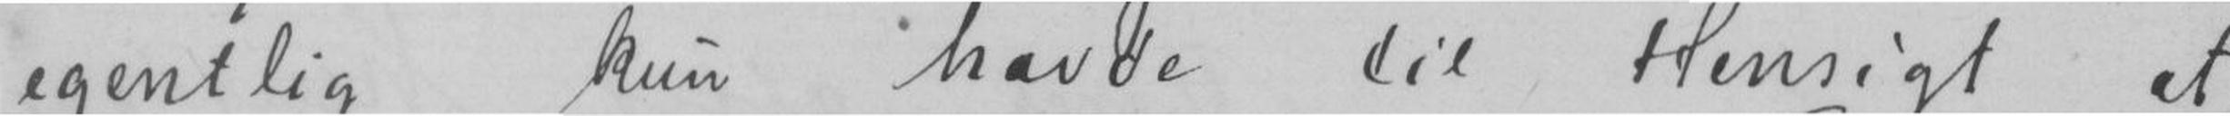egentlig kun havde til Hensigt at
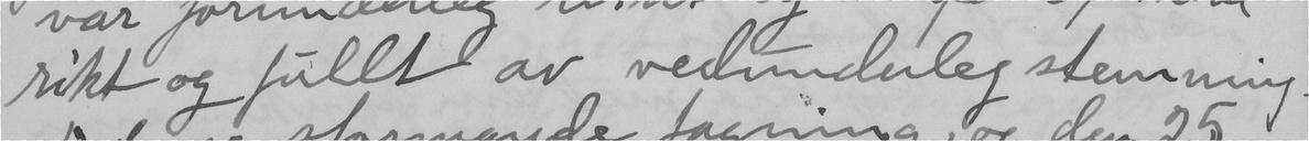rikt og fullt av vedunderleg stemning.
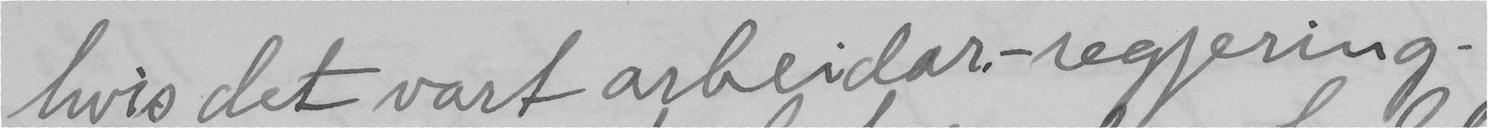hvis det vart arbeidar-regjering.
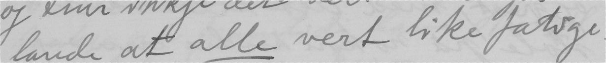lande at alle vert like fatige –
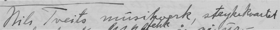Nils Tveits musikverk, strykekvartet
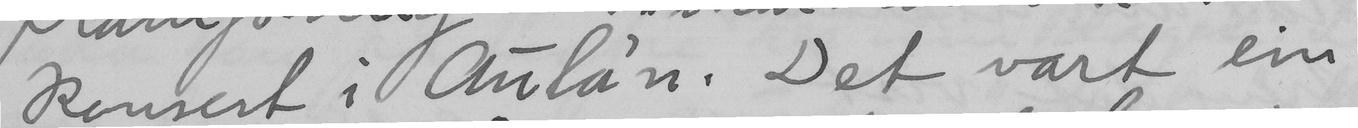konsert i Aula'n. Det vart ei
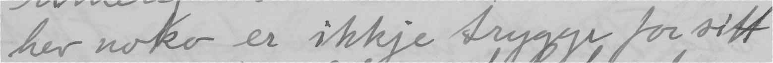hev noko er ikkje trygge for sitt
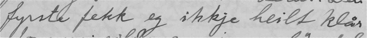fyrste fekk eg ikkje heilt klår
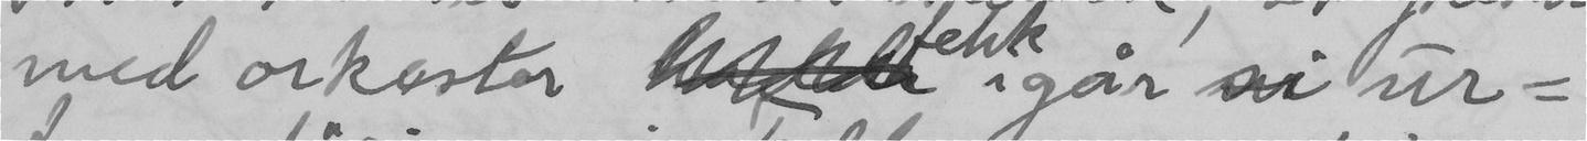med orkester fekk igår ur-
